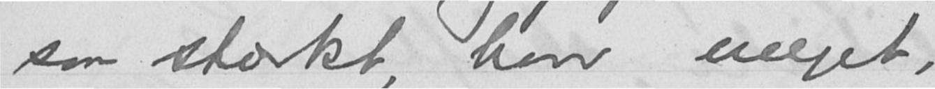saa stærkt, hvor meget,
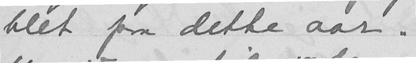blit paa dette aar.
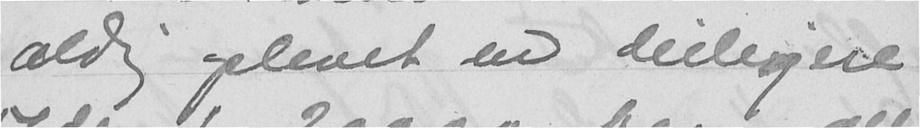aldrig oplevet en deiligere
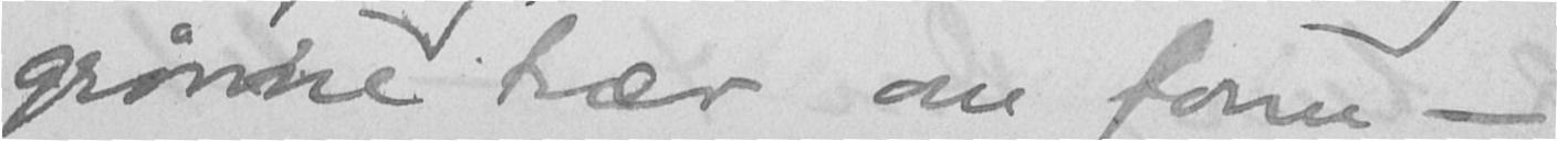grønne trær om form -
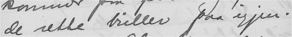de rette briller paa igjen.
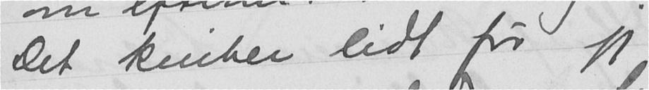Det kniber lidt før jeg
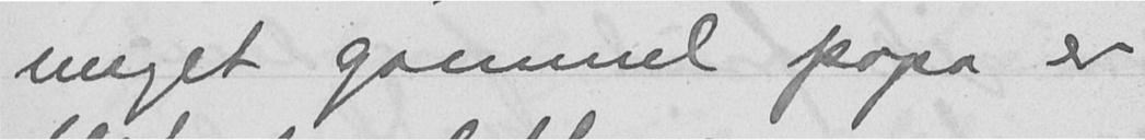meget gammel papa er
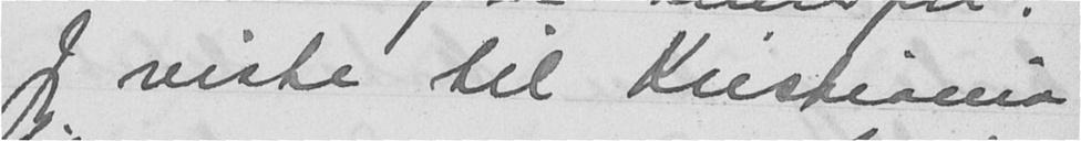Jeg reiste til Kristiania
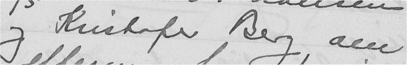og Kristofer Berg om
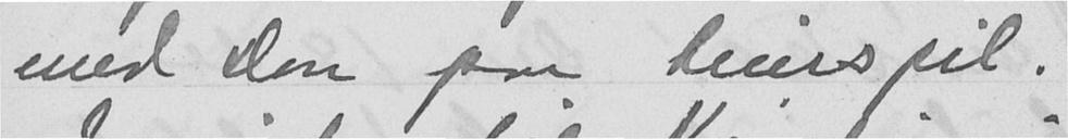med Don paa tenispil.
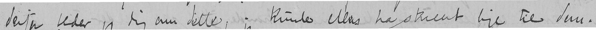derfor beder jeg dig om dette, jeg kunde ellers ha skrevet lige til dem.
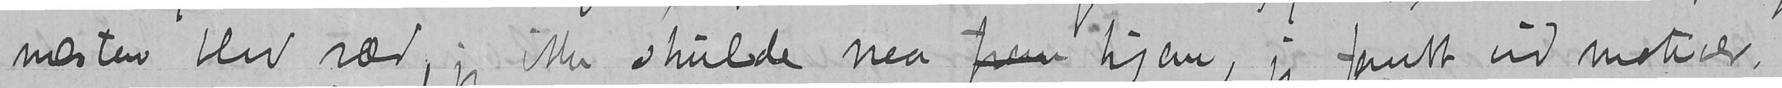næsten blev ræd, jeg ikke skulde naa paa hjem, jeg fandt ud motiver.
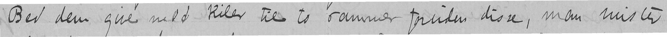Bed dem give med kiler til to rammer foruden disse, man mister
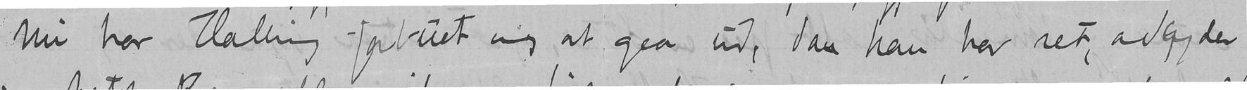Nu har Halling forbudt mig at gaa ud, dan han har ret, adlyder
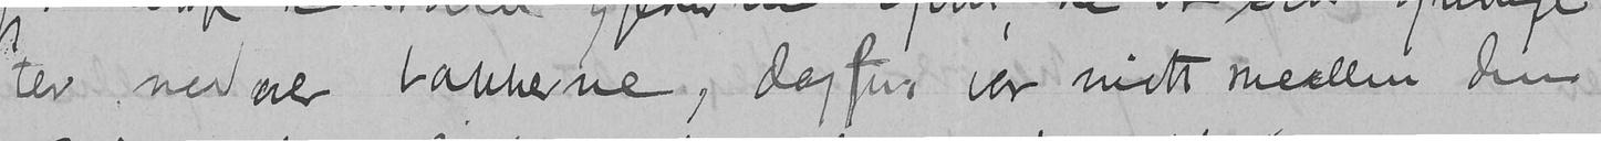ter nedover bakkerne, Dagfin var midt mellem dem
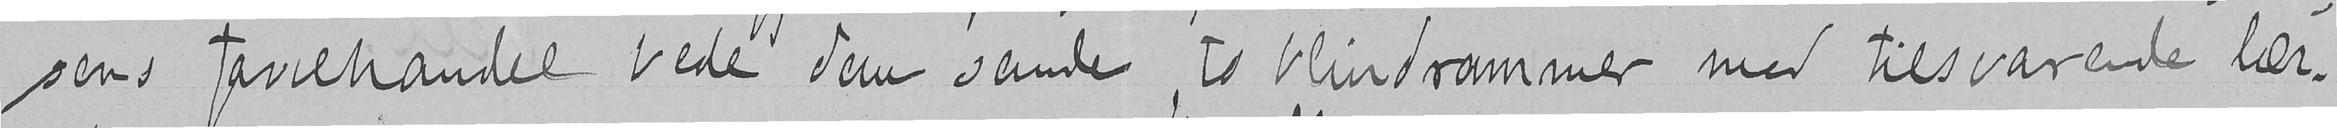sens farvehandel bede dem sende to blindrammer med tilsvarende lær-
